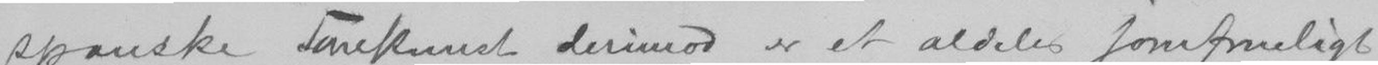spanske Tonekunst derimod er et aldeles jomfrueligt
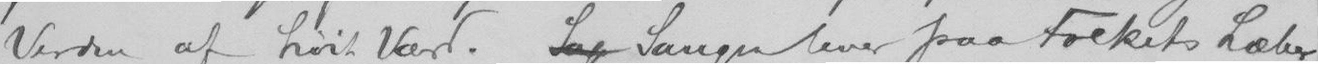Verden af Høit Værd. Sag Sangen lever paa Folkets Læber
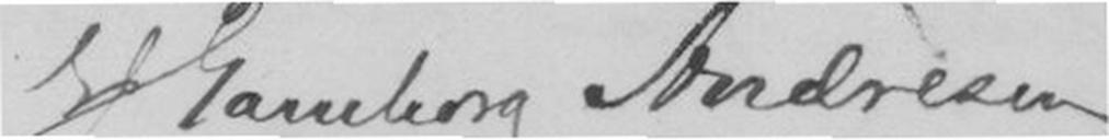E J Gamborg Andresen
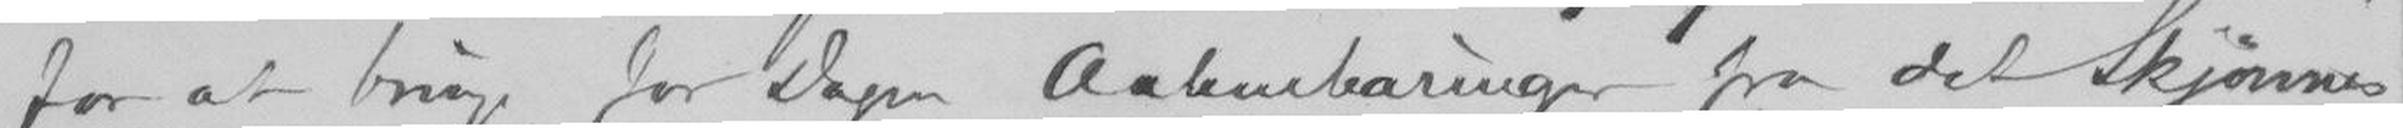for at bringe for dagen Aabenbaringer fra det Skjønnes
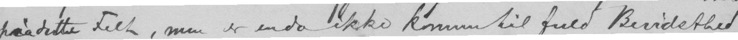paa dette Felt, men er en da ikke kommet til fuld Bevidsthed
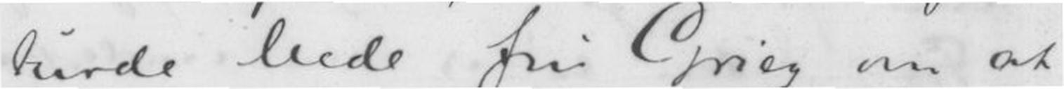turde bede fru Grieg om at
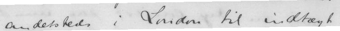andetsteds i London til indtægt
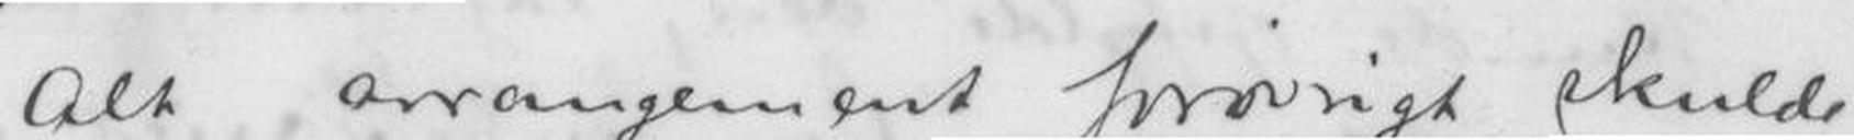Alt arragement forøvrigt skulde
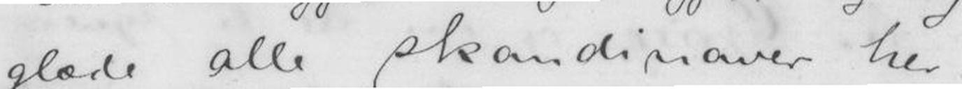glæde alle skandinaver her.
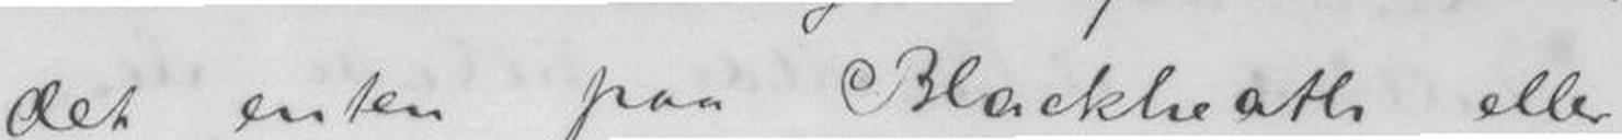det enten paa Blackheath eller
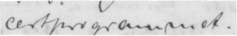certprogrammet
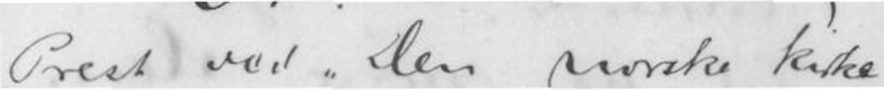Prest ved Den norske kirke
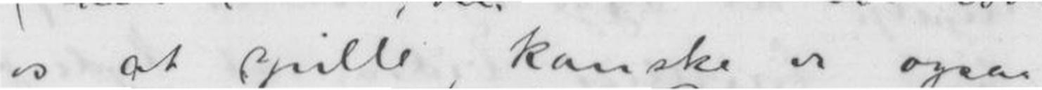os at spille, kanske vi ogsaa
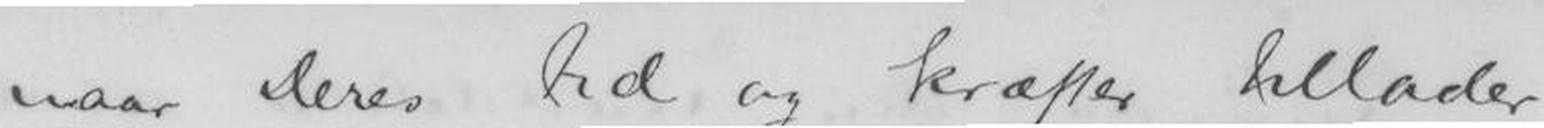naar Deres tid kræfter tillader
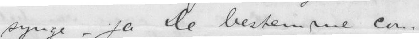synge - ja De bestemmer con-
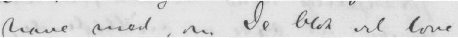have med, om De blot vil love
